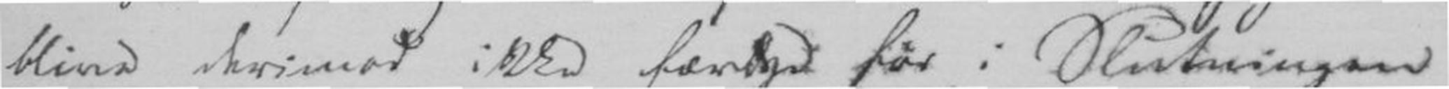blive derimod ikke færdig før i Slutningen
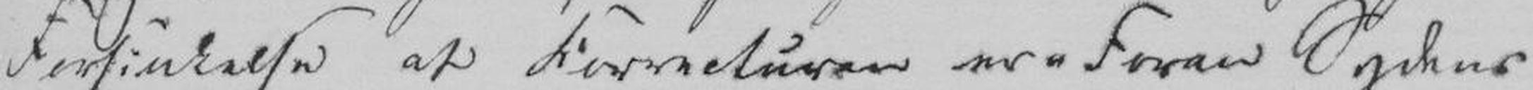Forsinkelse af Correcturen er "Foran Sydens
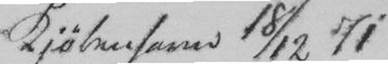Kjøbenhavn 18/12 71
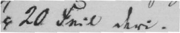c 20 Feil deri. -
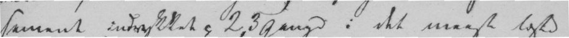sement indrykket c 2, 3 Gange i det meget læste
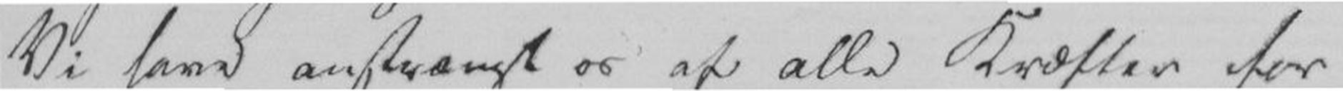Vi have anstrængt os af alle Kræfter for
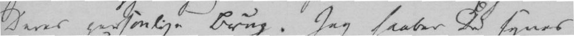Deres personlige Brug. Jeg haaber De synes
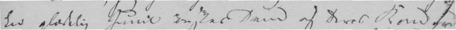En Glædelig Juul ønskes Dem og Deres Kone fra
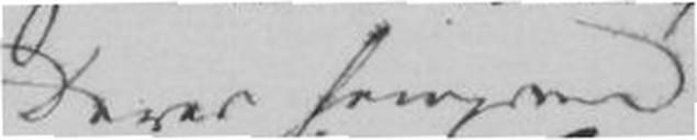Deres hengivne
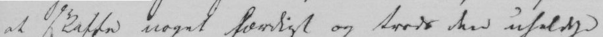at Skaffe noget færdigt og trods den uheldige
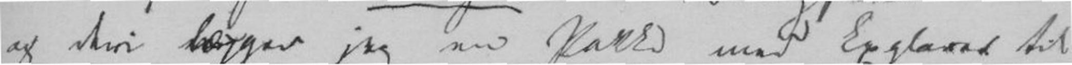og deri lægger jeg en Pakke med Explarer til
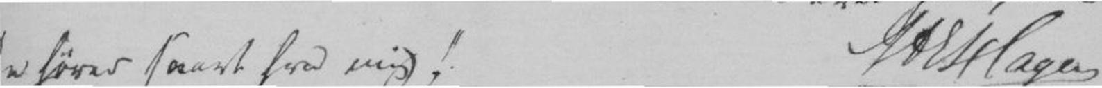De hører snart fra mig! S A E Hagen
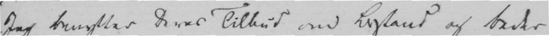Jeg benytter Deres Tilbud om Bistand og beder
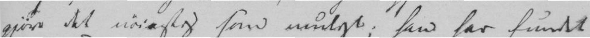gjøre det nøiagtig som muligt; han har fundet
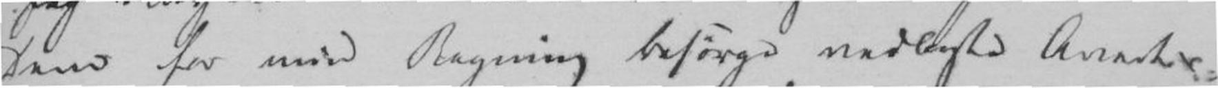Dem for min Regning besørge vedlagte Avertis-
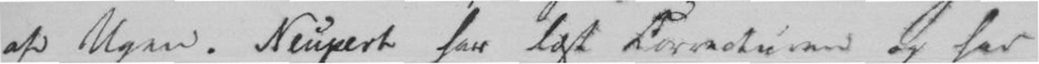af Ugen. Neupert har læst Correcturen og har
